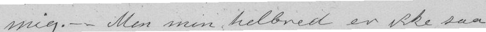mig.-- Men min helbred er ikke saa
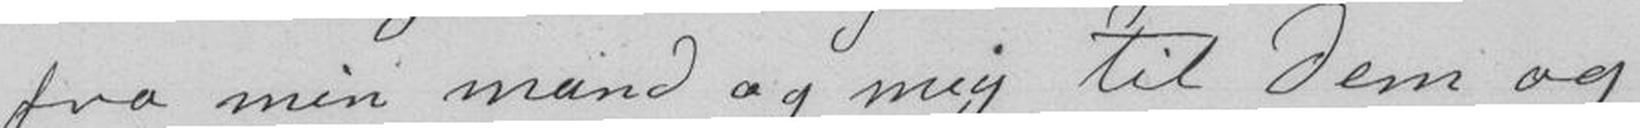fra min mand og mig til Dem og
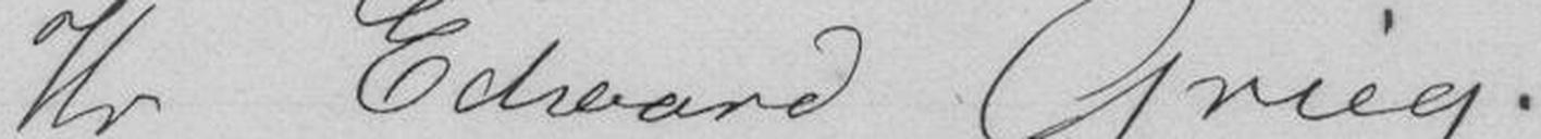Hr Edvard Grieg.
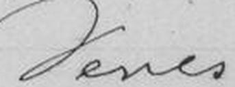Deres
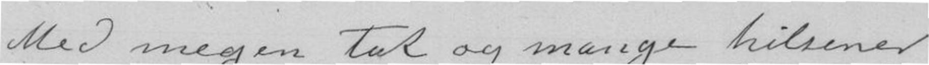Med megen Tak og mange hilsener
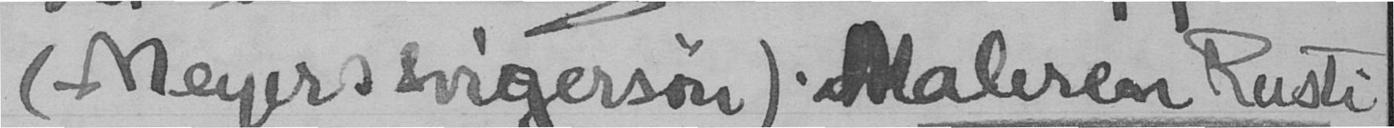(Meyers svigersøn). Maleren Rusti
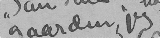gaarden, jeg
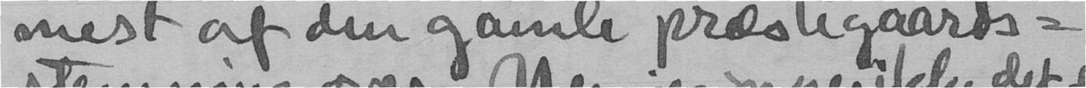mest af den gamle præstegaards-
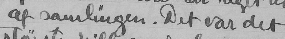af samlingen. Det var det
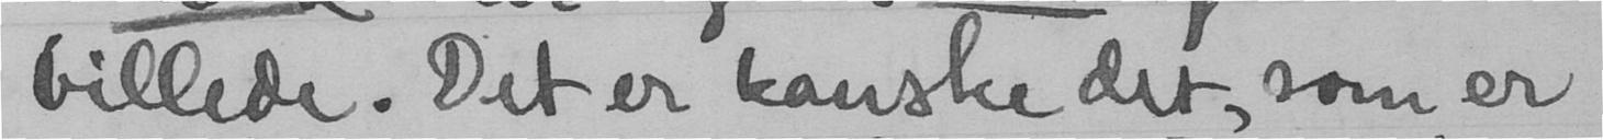billede. Det er kanske det, som er
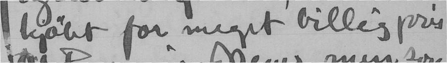kjøbt for meget billig pris
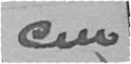cm
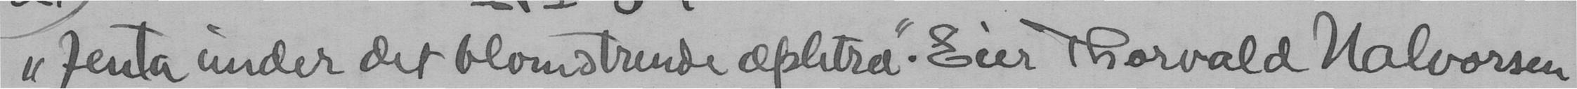"Jenta under det blomstrende æpletret". Eier Thorvald Halvorsen
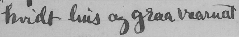hvidt hus og graa vaarnat
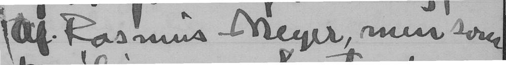af Rasmus Meyer, men som
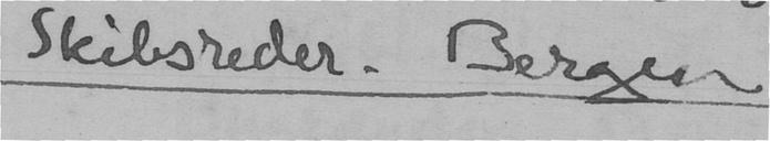Skibsreder. Bergen
